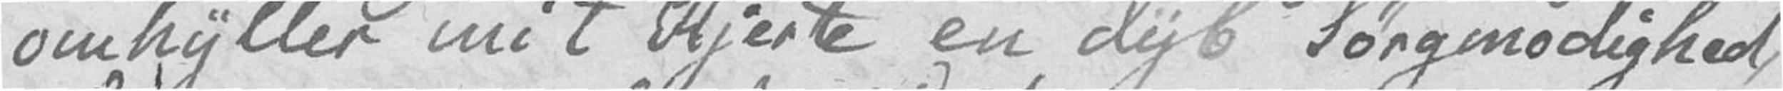omhyller mit Hjerte en dyb Sørgmodighed,
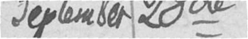September 28de
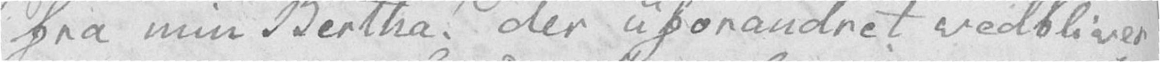fra min Bertha! der uforandret vedbliver
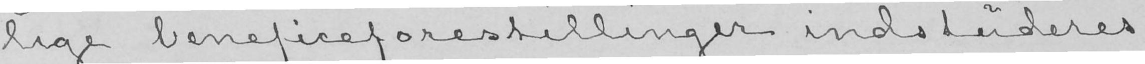lige beneficeforestillinger indstuderes,
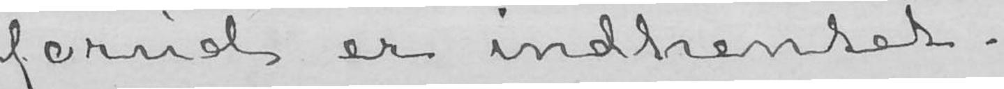forud er indhentet.
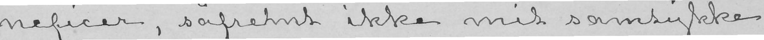neficer, såfremt ikke mit samtykke
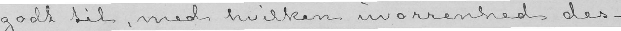godt til, med hvilken uvorrenhed des-
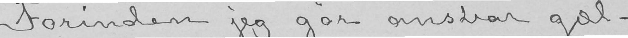Forinden jeg gør ansvar gæl-
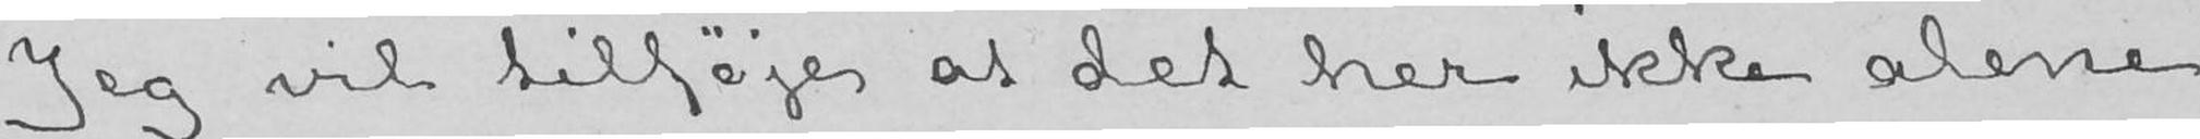Jeg vil tilføje at det her ikke alene
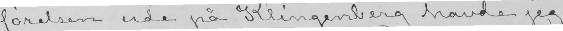førelsen ude på Klingenberg havde jeg
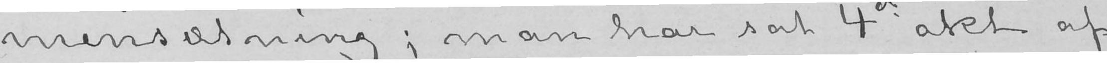mensætning; man har sat 4de akt af
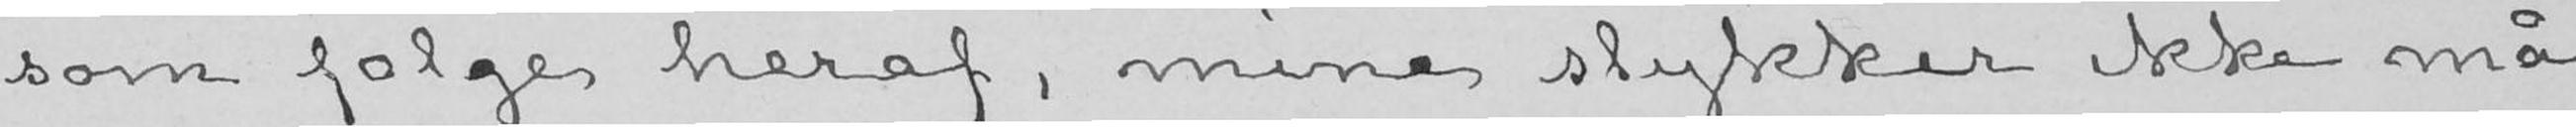som følge heraf, mine stykker ikke må
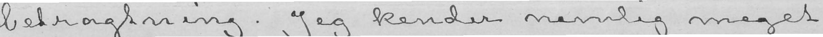betragtning. Jeg kender nemlig meget
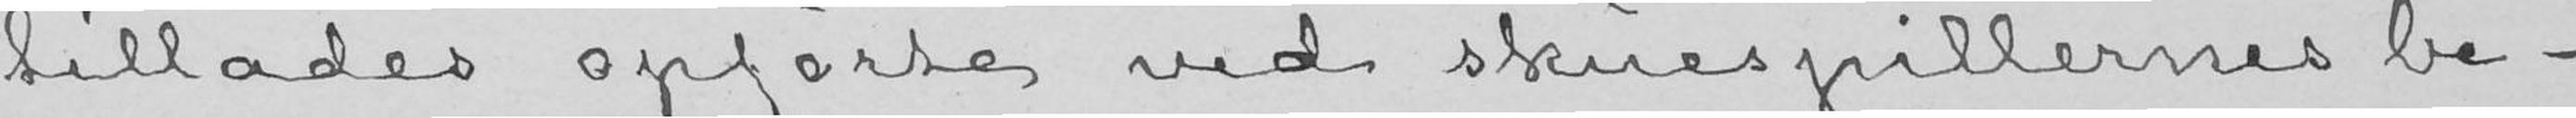tillades opførte ved skuespillernes be-
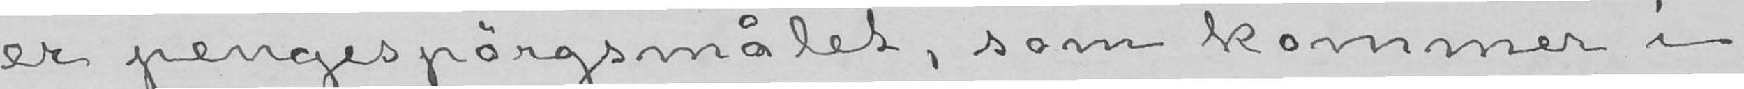er pengespørgsmålet, som kommer i
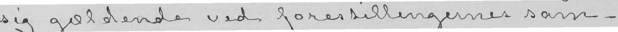sig gældende ved forestillingernes sam-
